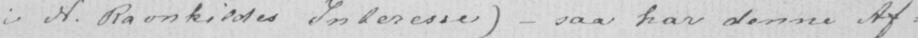i N. Ravnkildes Interesse)- saa har denne Af-
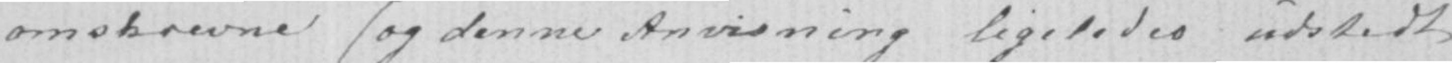omskrevne (og denne Anvisning ligeledes udstedt
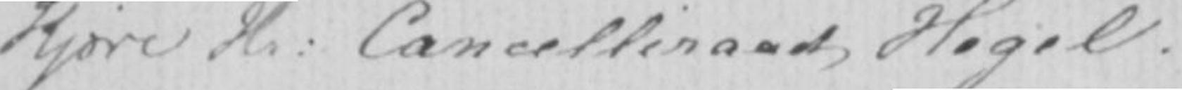Kjære Hr: Cancelliraad Hegel!
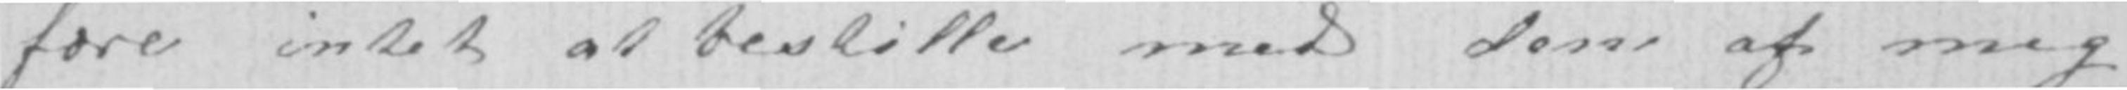fære intet at bestille med den af mig
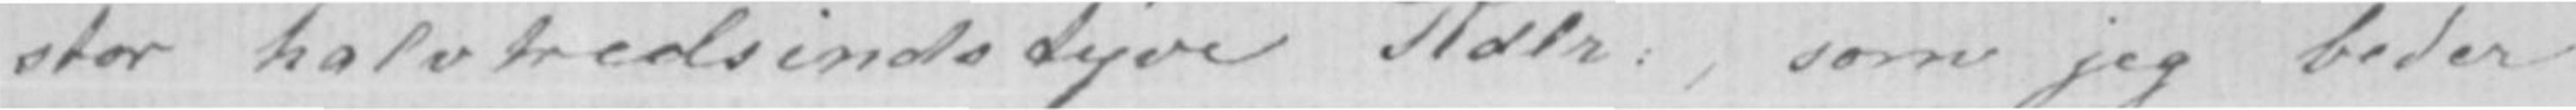stor halvtredsindstyve Rdlr:, som jeg beder
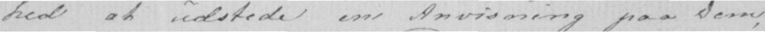hed at udstede en Anvisning paa Dem,
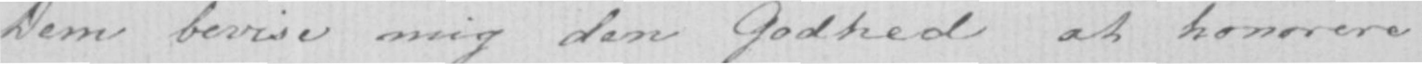Dem bevise mig den Godhed at honorere.
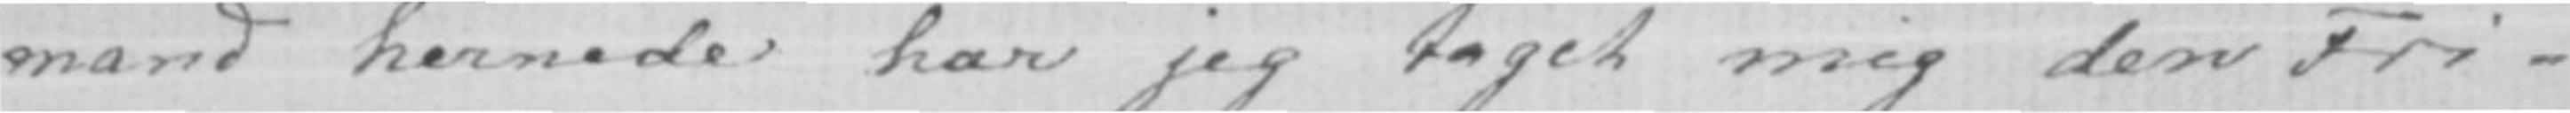mand hernede har jeg taget mig den Fri-
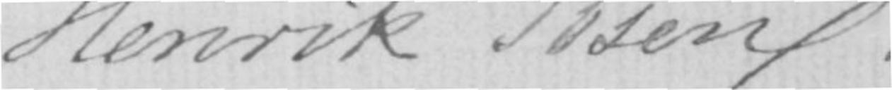Henrik Ibsen.
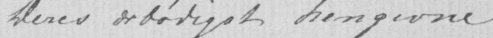Deres ærbødigst hengivne
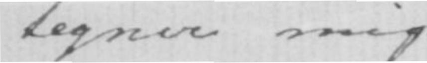tegner mig
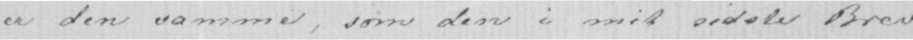er den samme, som den i mit sidste Brev
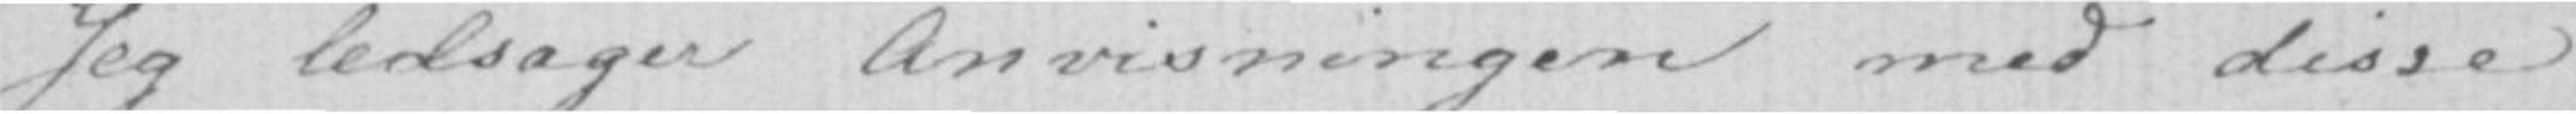Jeg ledsager Anvisningen med disse
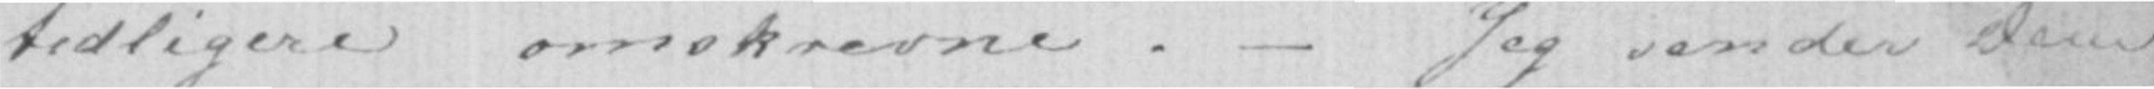tidligere omskrevne.- Jeg sender Dem
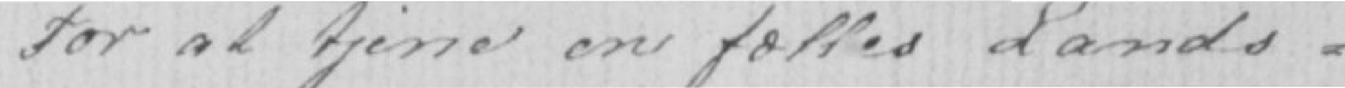For at tjene en fælles Lands-
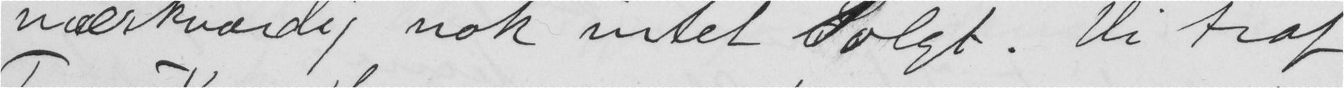merkværdig nok intet Solgt. Vi traf
